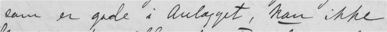som er gode i Anlægget, kan ikke
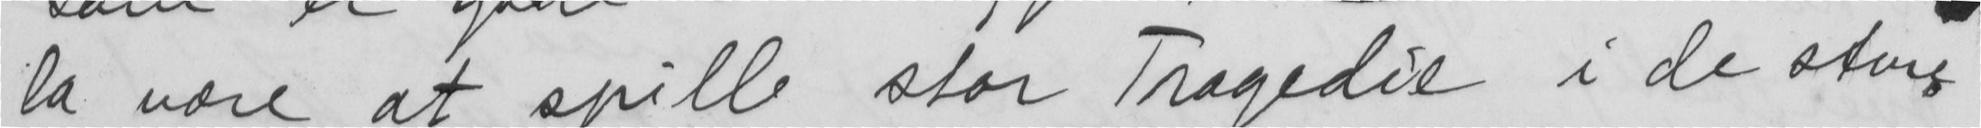la være at spille stor Tragedie i de store
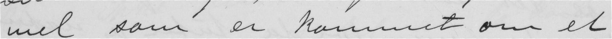mel som er kommet om et
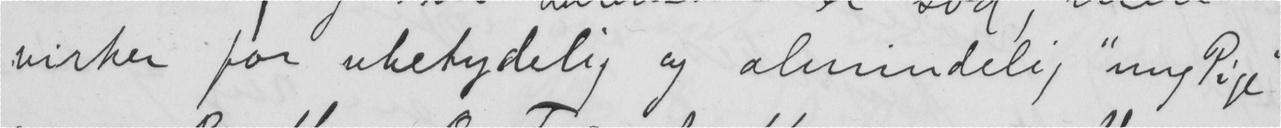virker for ubetydelig og almindelig "ung Pige"
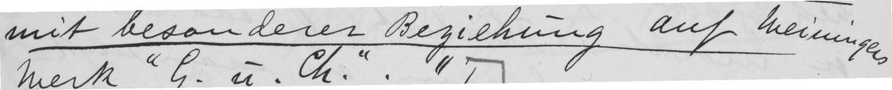mit besonderer Begiehing auf Meiningen.
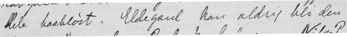hele haabløst. Eldegard kan aldrig bli den
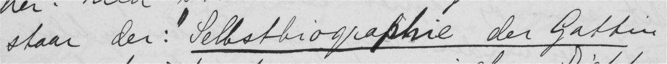staar der: "Selbstbiographie der Gattire
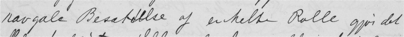ravgale Besættelse af enkelte Rolle gjør det
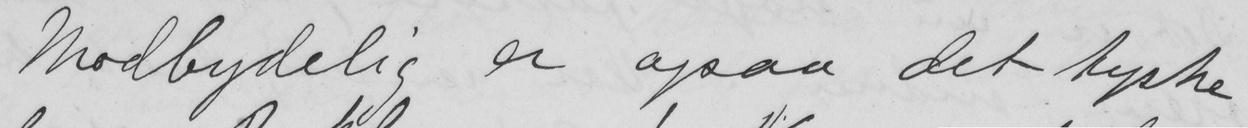Modbydelig er ogsaa det tyske
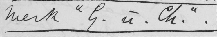Merk "G. u. Ch.".
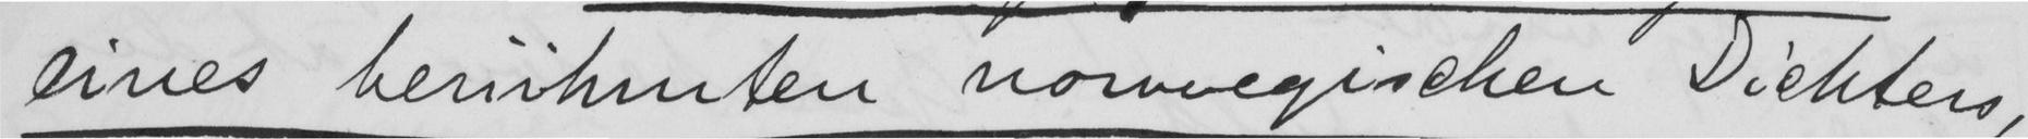eines beruhinten norviegischen Diekters
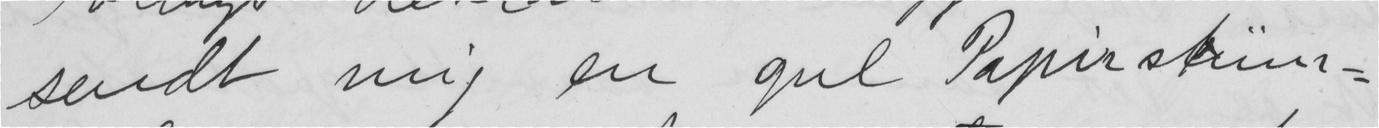sendt mig en gul Papirstrim-
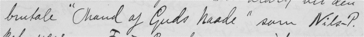brutale "Mand af Guds Naade" som Nils-P.
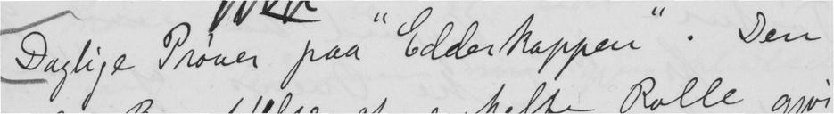Daglige Prøver paa "Edderkoppen". Den
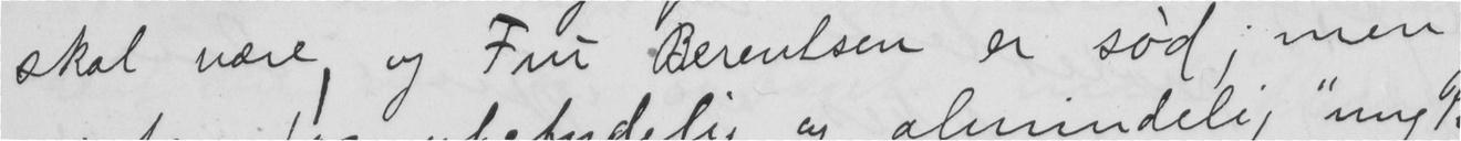skal være og Fru Berentsen er sød; men
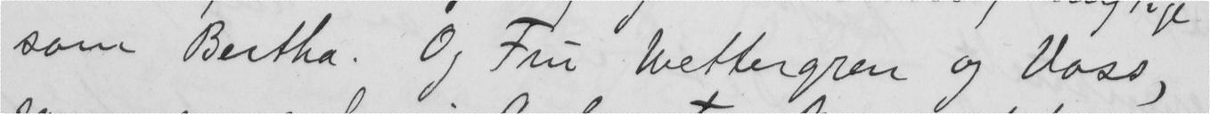som Bertha. Og Fru Wettergren og Voss,
